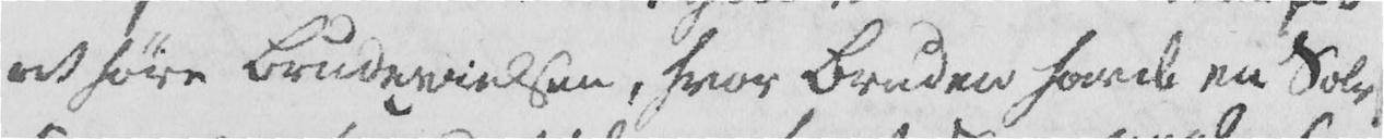at høre Brudevielsen, hvor Bruden havde en Sølv
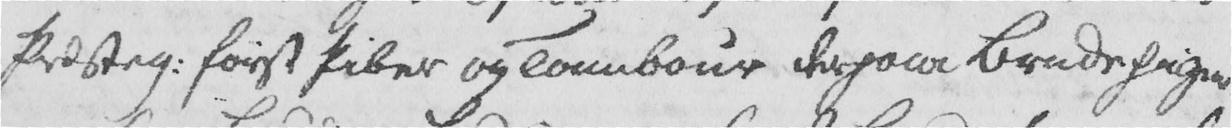Præsteg. først Piber og Tambour derpaa Brudepiger-
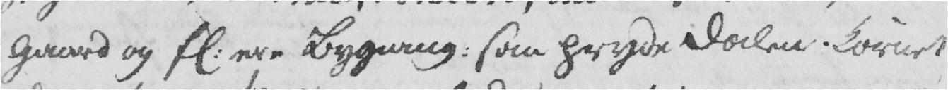Gaard og fl. ere Bygning. som pryde Dalen. Kornet
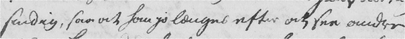sindig, saa at han jo længes efter at see andre
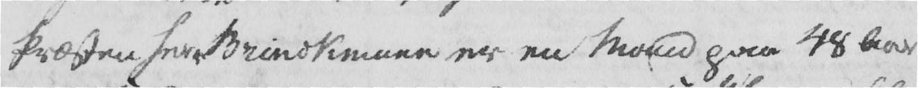Præsten her Brinckmann er en Mand paa 48 Aar
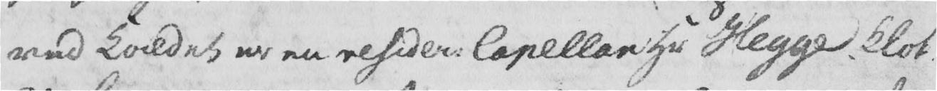ved Kaldet er en resider Capellan Hr Hegge. klok.
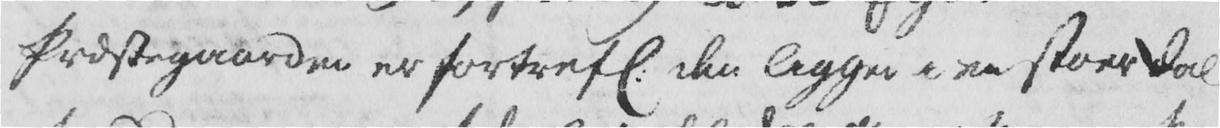Præstegaarden er fortrefl. den ligge i en stoer dal
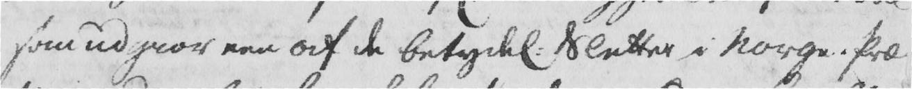som udgiør een af de betydel. Sletter i Norge. Præ-
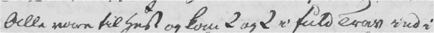Alle vare til Hest og kom 2 og 2 i fuld Trav ind i
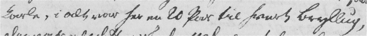Karle, i alt var her en 20 Par til hvert Bryllup,
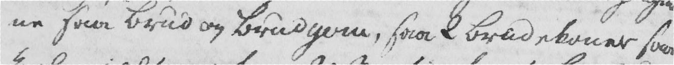ne saa Brud og Brudgom, saa 2 Brudekoner saa
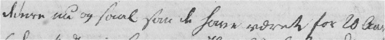de ere nu og saal som de have været for 20 Aar
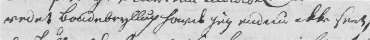ved et Bondebryllup havde jeg endnu ikke seet,
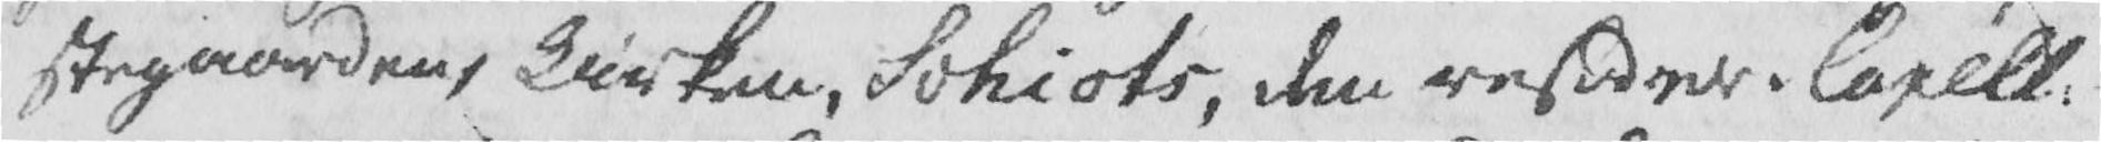stegaarden, Kirken, Schiots, den resider. Capell.
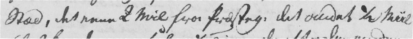Stæd, det eene 2 Mil fra Præsteg. det andet 1/2 Miil
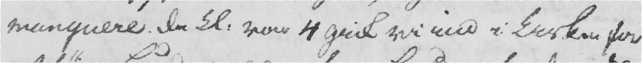manquere. Da kl. var 4 gick vi ind i Kirken for
